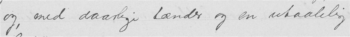og, med daarlige tænder og en utaalelig
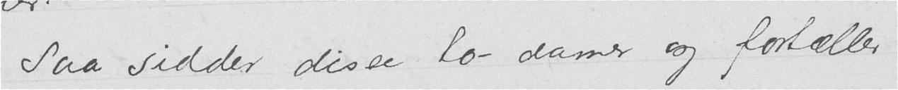— Saa sidder disse to damer og fortæller
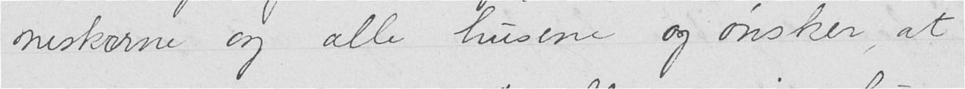neskerne og alle husene og ønsker, at
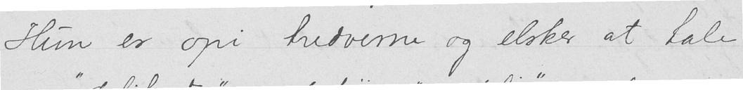Hun er opi tredverne og elsker at tale
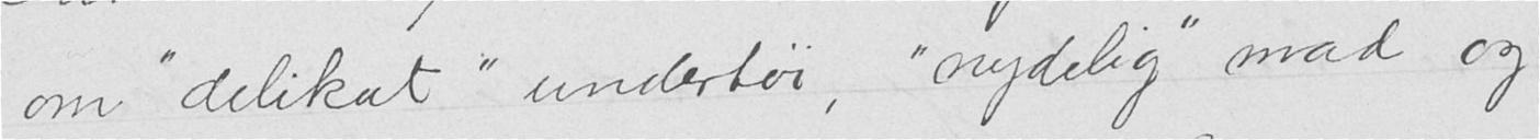om "delikat" undertøi, "nydelig" mad og
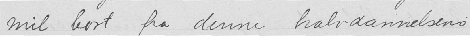mil bort fra denne halvdannelsens
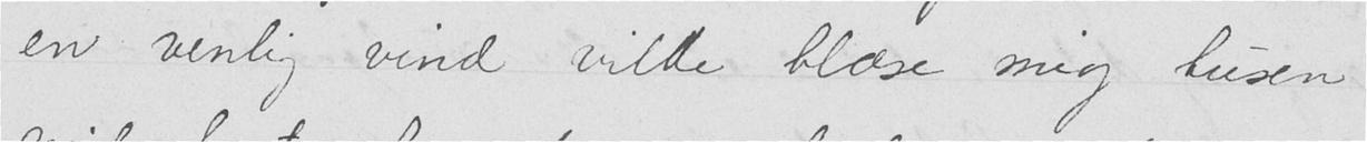en venlig vind vilde blæse mig tusen
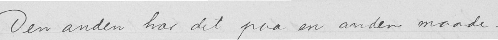Den anden har det paa en anden maade.
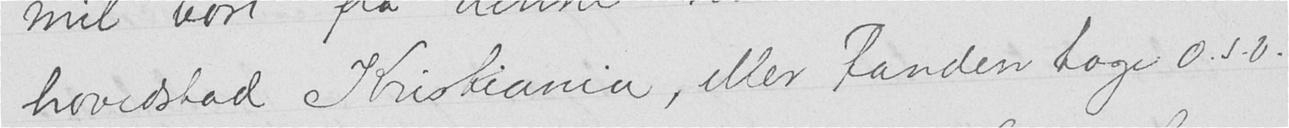hovedstad Kristiania, eller Fanden tage o.s.v.
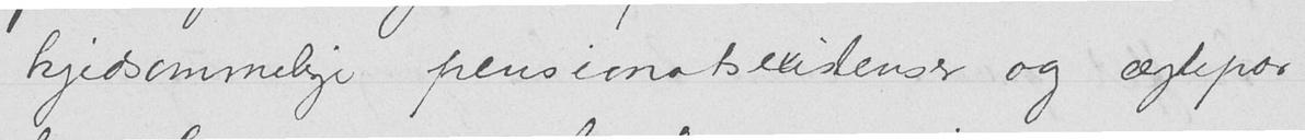kjedsommelige pensionatsexistenser og ægtepar
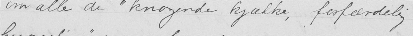om alle de "knagende kjække, forfærdelig
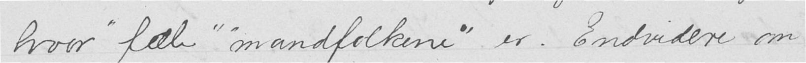hvor "fæle" "mandfolkene" er. Endvidere om
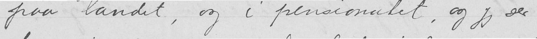paa landet, og i pensionatet, og jeg ser
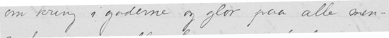om kring i gaderne og glor paa alle men-
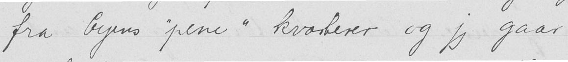fra byens "pene" kvarterer og jeg gaar
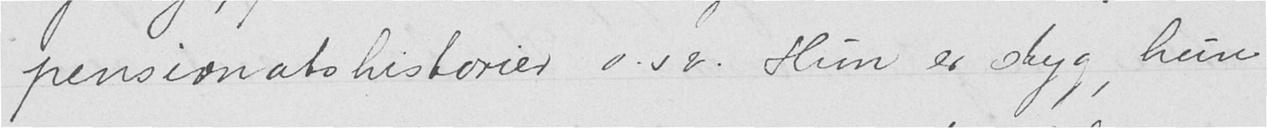pensionatshistorier o.s.v. Hun er styg, hun
